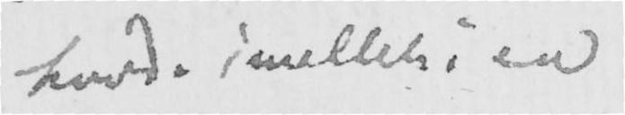kand. Smeltet i en
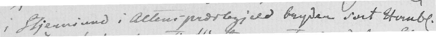i Stjernsund i Altens præstegjeld bryder sort Hornbl.
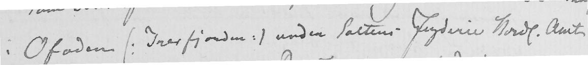i Ofoden /: Tverfjorden :/ under Saltens Fogderie Nordl. Amt
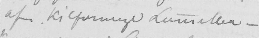af kilformage Lumeller -
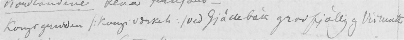Kongsgrunden /: Kongsværket :/ ved Gjällebäk gror fjalig og Vimatte
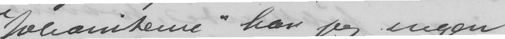"Johaniterne" har jeg ingen
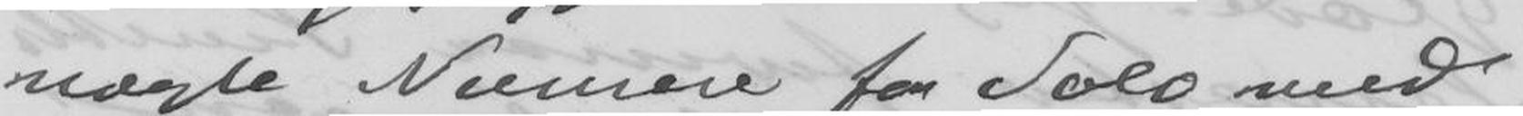nogle Numere for Solo med
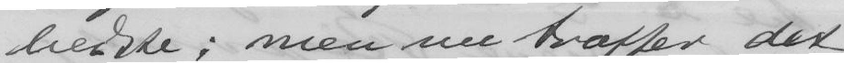bedste; men nu træffer det
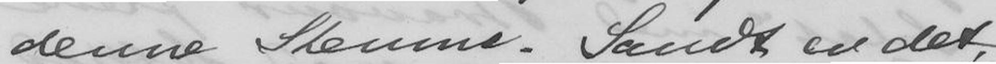denne Stemme. Sandt er det
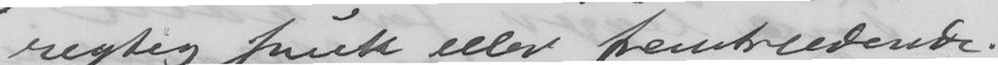rigtig smuk eller fremtredende.
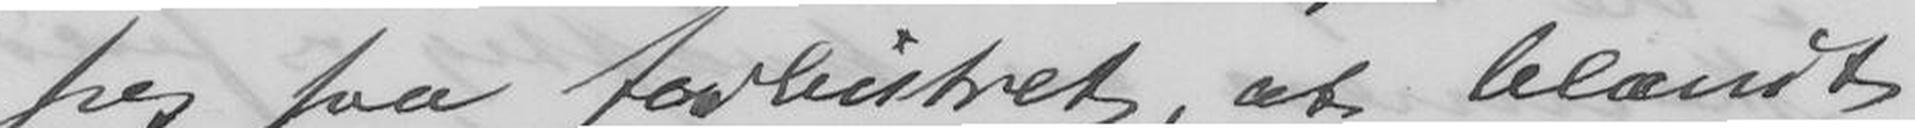sig saa forbistet, at blandt
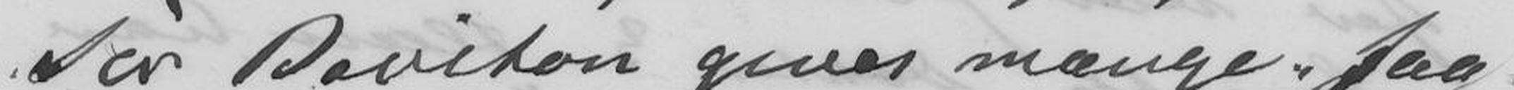For Bariton gives mange saa
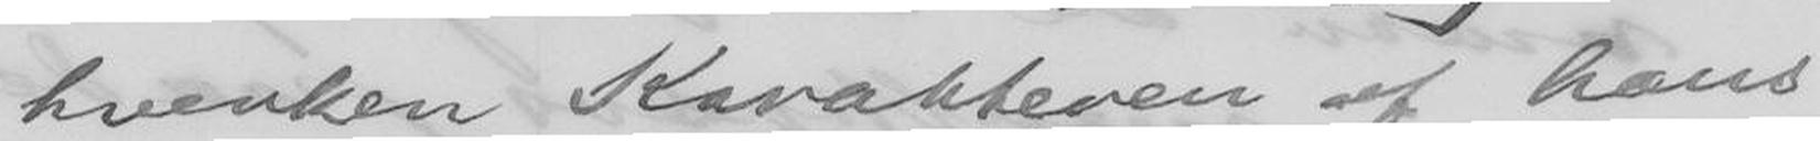hverken Karakteren af hans
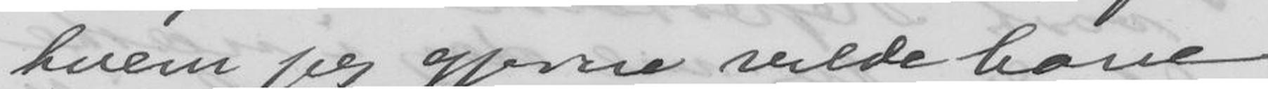hvem jeg gjerne vilde have
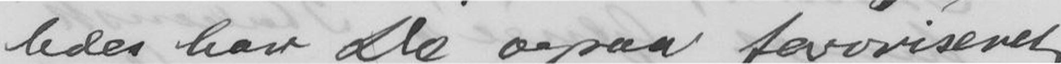ledes har De ogsaa favoriseret
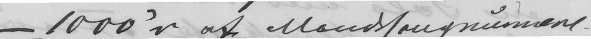- 1000'r af Mandssangnumere.
